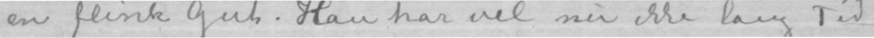en flink Gut. Han har vel nu ikke lang Tid
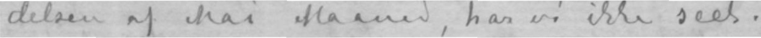delsen af Mai Maaned, har vi ikke seet.
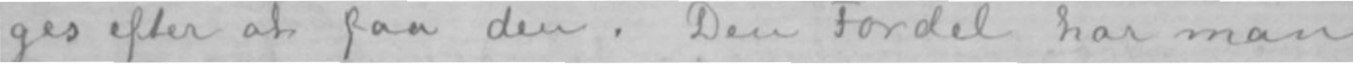ges efter at faa den. Den Fordel har man
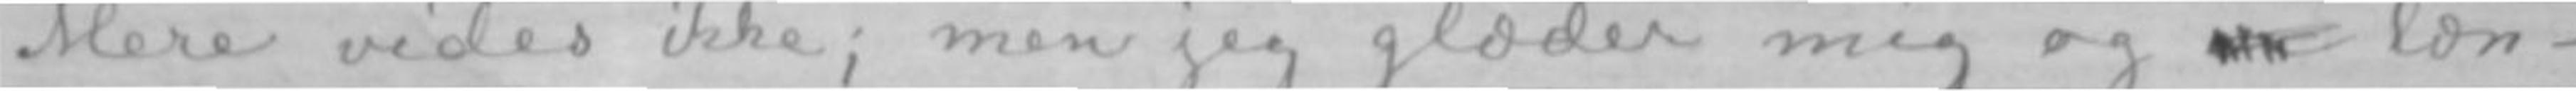Mere vides ikke; men jeg glæder mig og  læn-
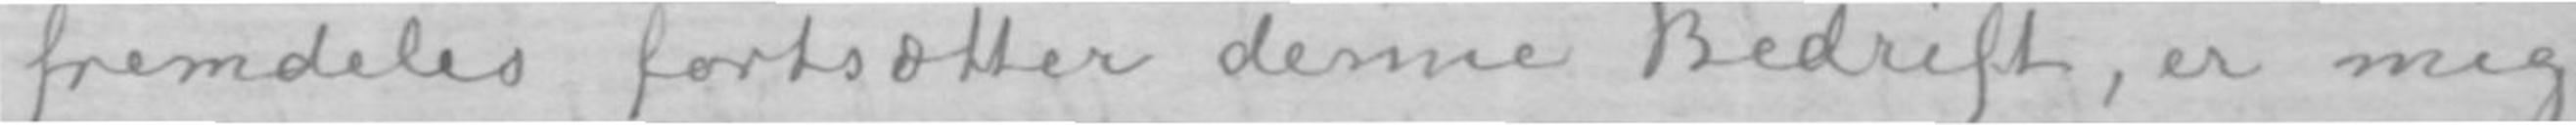fremdeles fortsætter denne Bedrift, er mig
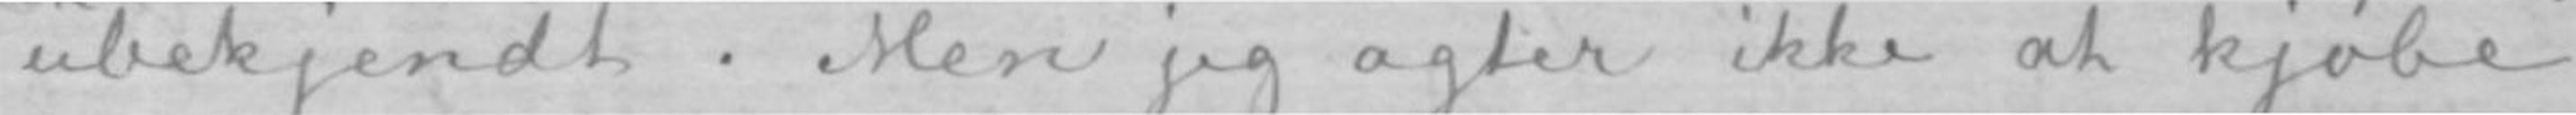ubekjendt. Men jeg agter ikke at kjøbe
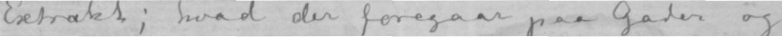Extrakt; hvad der foregaar paa Gader og
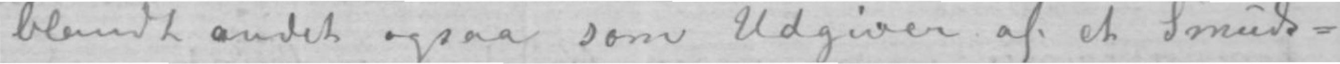blandt andet ogsaa som Udgiver af et Smuds-
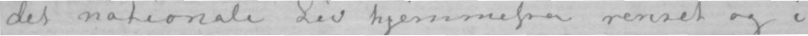det nationale Liv hjemmefra renset og i
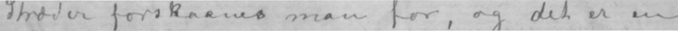Stræder forskaanes man for, og det er en
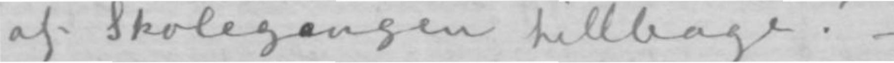af Skolegangen tillbage?-
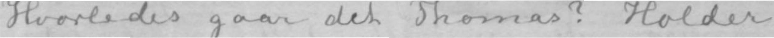Hvorledes gaar det Thomas? Holder
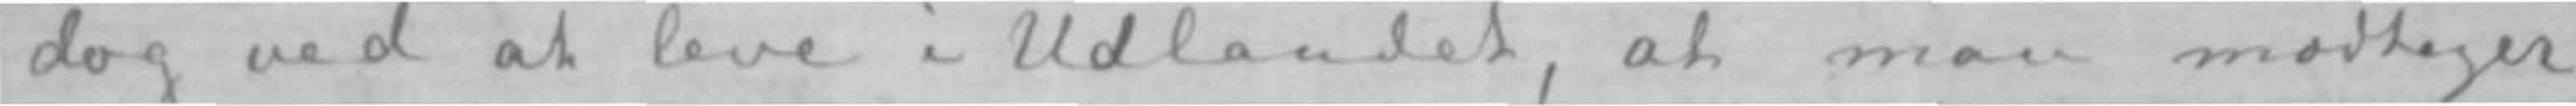dog ved at leve i Udlandet, at man modtager
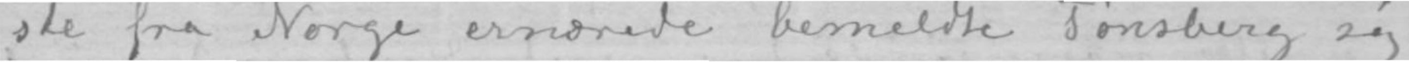ste fra Norge ernærede bemeldte Tønsberg sig
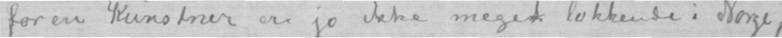for en Kunstner er jo ikke meget lokkende i Norge,
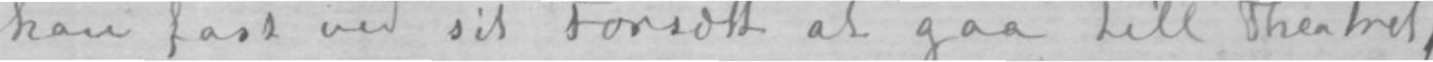han fast ved sit Forsætt at gaa till Theatret,
